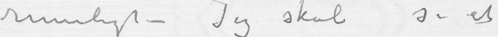rimeligt – Jeg skal se at
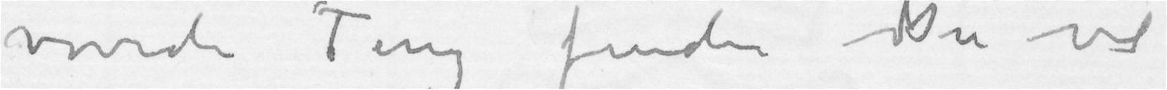vovede Ting finder Du vel
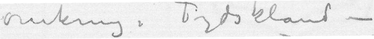omkring i Tydskland –
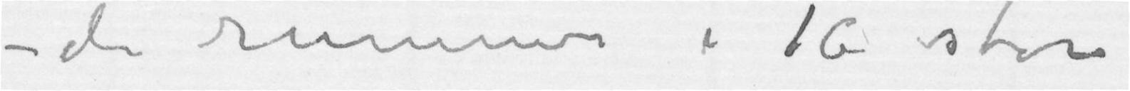– de rummes i 16 store
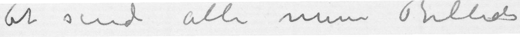At sende alle min Billeder
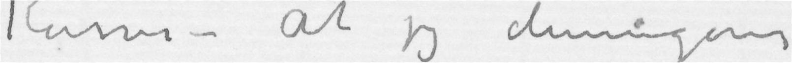Kasser – At jeg dennegang
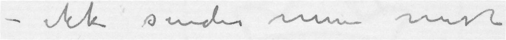– ikke sender mine mest
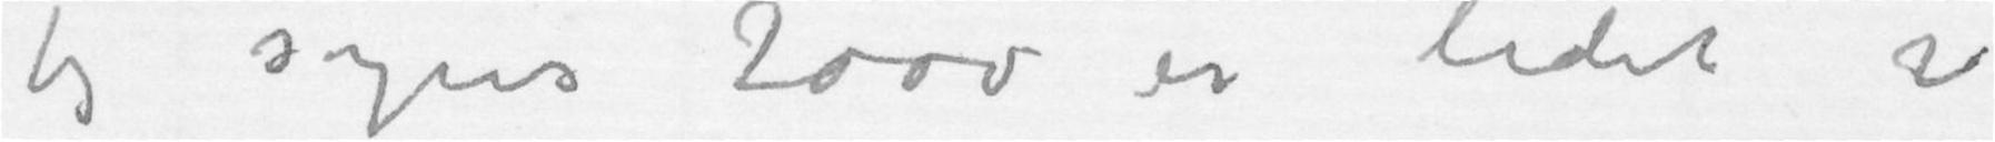jeg syns 2000 er lidet
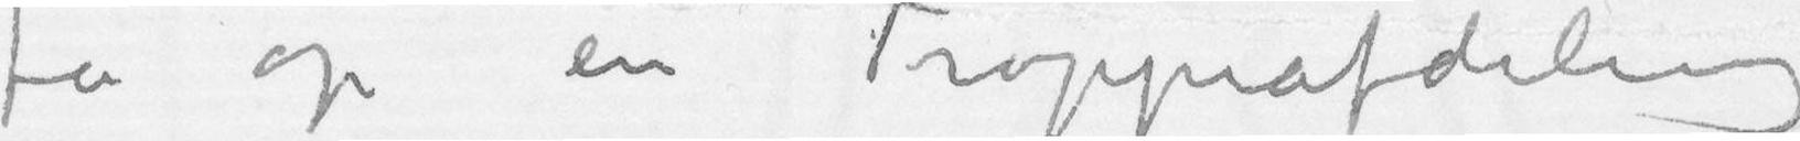ta op en Troppeafdeling
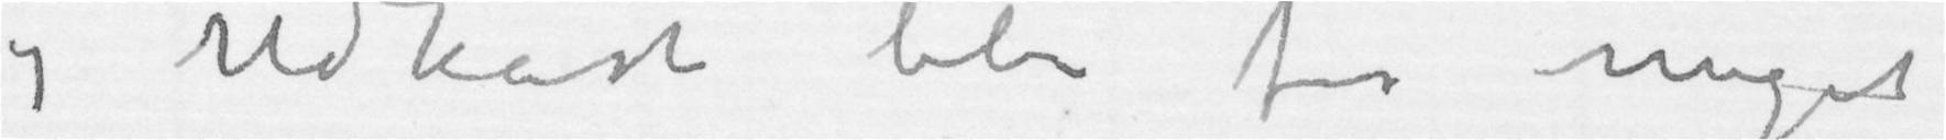og Udkast blir for meget
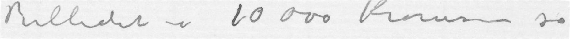Billedet i 10 000 Kroner så
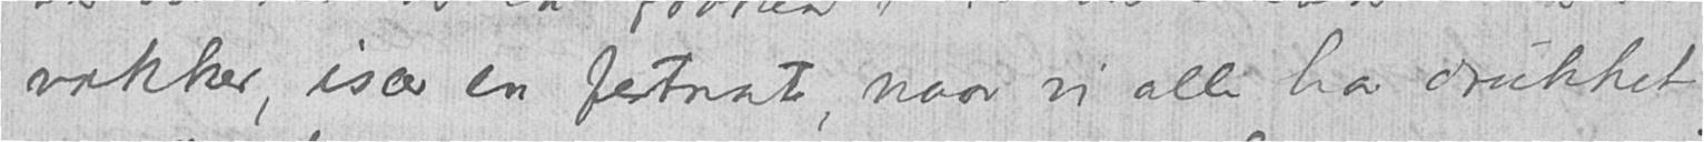vakker, især en festnat, naar vi alle har drukket
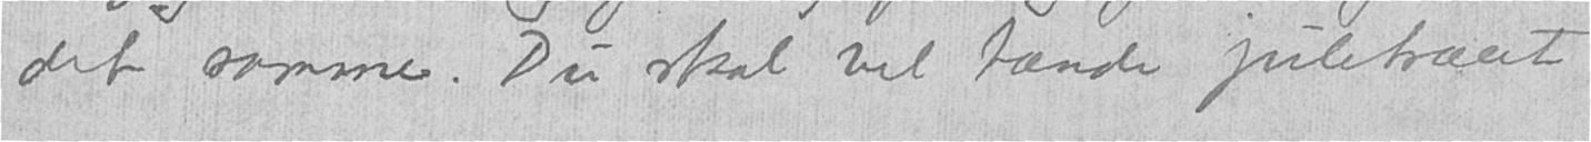det samme. Du skal vel tænde juletræet
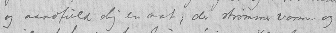og aandfuld slig en nat; der strømmer varme og
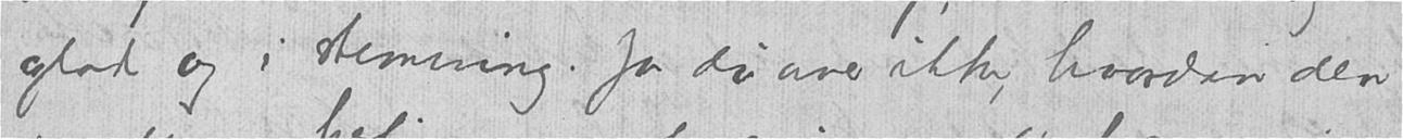glad og i stemning. Ja du aner ikke, hvordan den
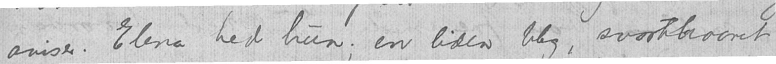aviser. Elena hed hun; en liden bleg, svarthaaret
